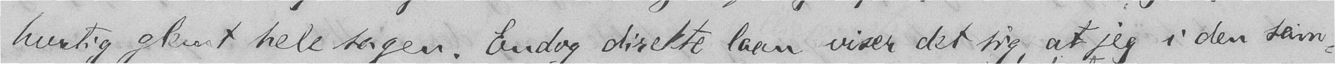hurtig glemt hele sagen. Endog direkte laan viser det sig, at jeg i den sam-
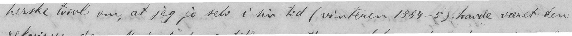herske tvivl om, at jeg jo selv i sin tid (vinteren 1884-5) havde været den
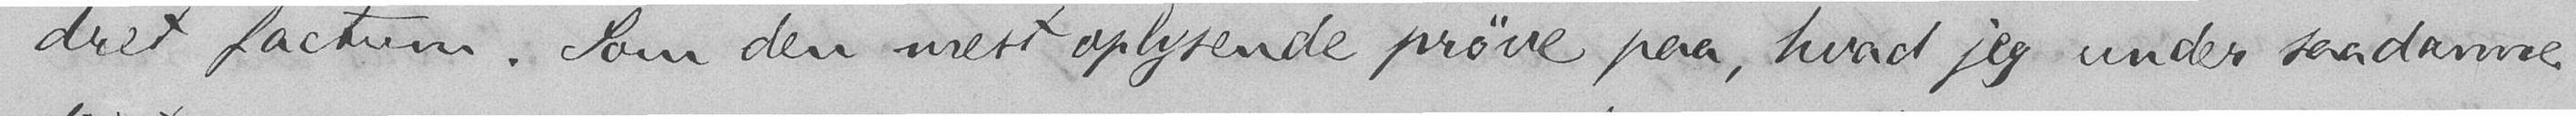dret factum. Som den mest oplysende prøve paa, hvad jeg under saadanne
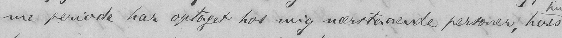me periode har optaget hos mig nærstaaende personer, hvis
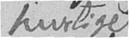hurtige
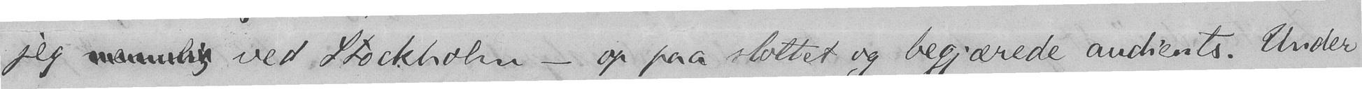jeg  ved Stockholm - op paa slottet og begjærede audients. Under
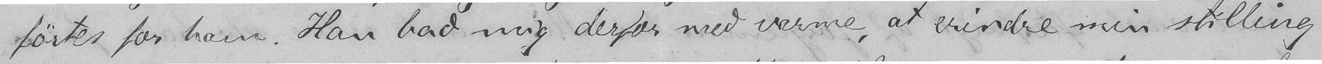førtes for ham. Han bad mig derfor med varme, at erindre min stilling
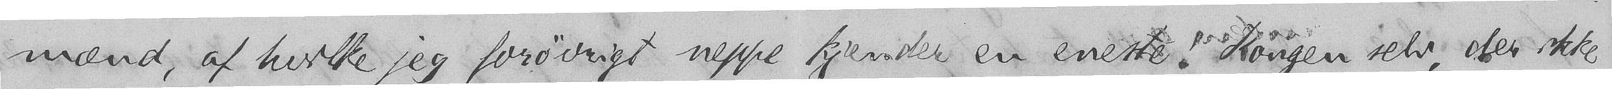mænd, af hvilke jeg forøvrigt neppe kjender en eneste! Kongen selv, der ikke
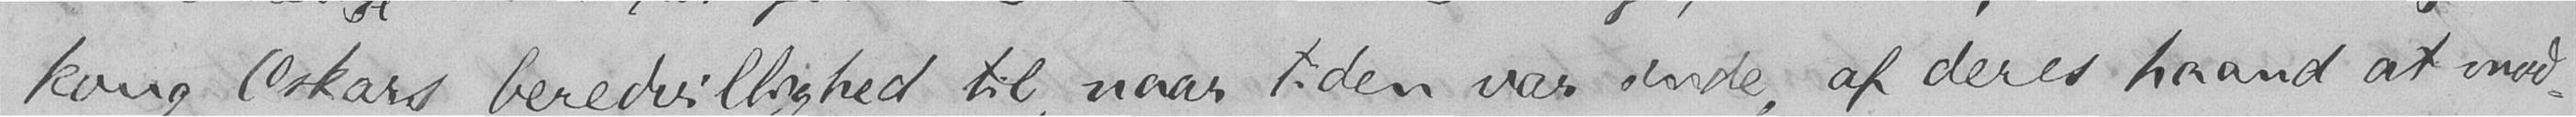kong Oskars beredvillighed til, naar tiden var inde, af deres haand at mod-
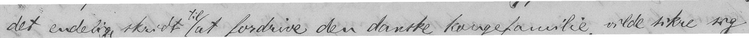det endelige skridt til at fordrive den danske kongefamilie, vilde sikre sig
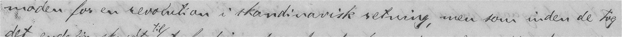moden for en revolution i skandinavisk retning, men som inden de tog
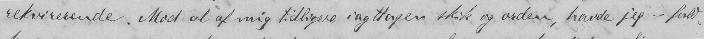rekvirerende. Mod al af mig tidligere iagttagen skik og orden, havde jeg - fuld-
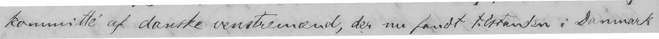kommitté af danske venstremænd, der nu fandt tilstanden i Danmark
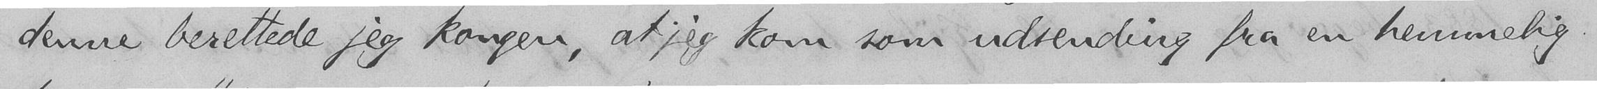denne berettede jeg kongen, at jeg kom som udsending fra en hemmelig
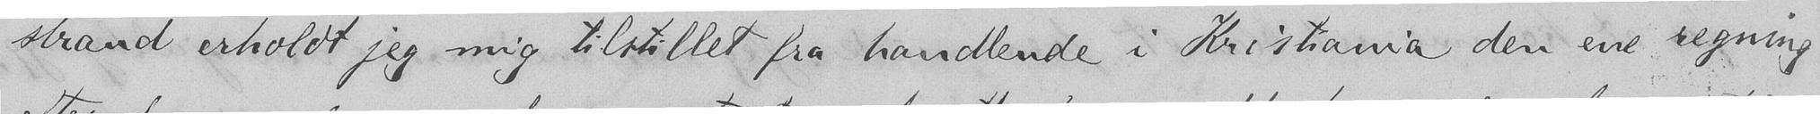strand erholdt jeg mig tilstillet fra handlende i Kristiania den ene regning
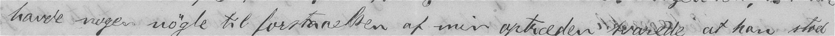havde nogen nøgle til forstaaelsen af min optræden, svarede, at han stod
